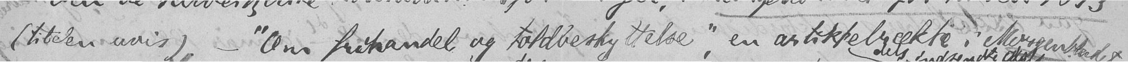(titelen uvis) - "Om frihandel og toldbeskyttelse", en artikkelrække i Morgenbladet
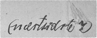(næstsidste?)
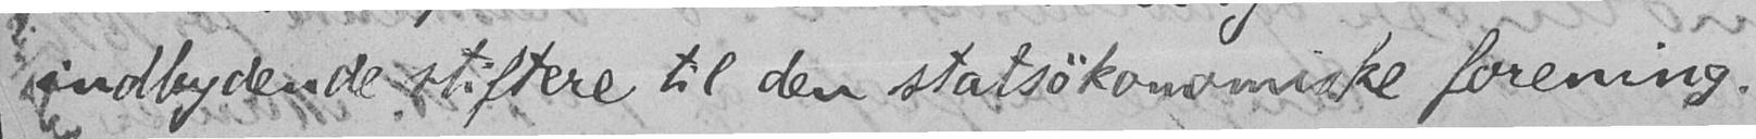indbydende stiftere til den statsøkonomiske forening.
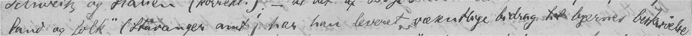land og folk" (Stavanger amt) har han leveret væsentlige bidrag til byernes beskrivelse,
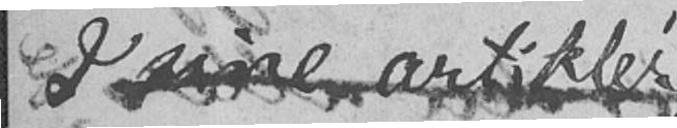I
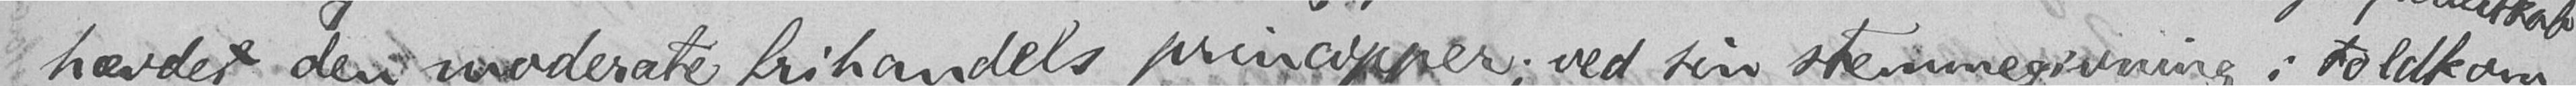hævdet den moderate frihandels principper; ved sin stemmegivning i toldkom-
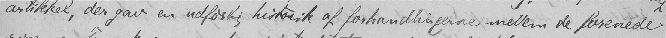artikkel, der gav en udførlig historik af forhandlingerne mellem de forenede
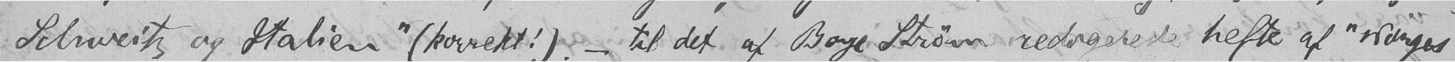Schweitz og Italien" (korrekt!); - til det af Boye Strøm redigerede hefte af "Norges
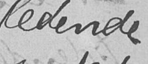ledende
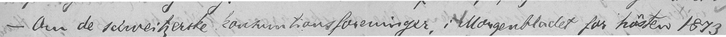- Om de schweitzerske konsumtionsforeninger, i Morgenbladet for høsten 1873
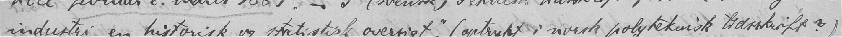industri, en historisk og statistisk oversigt", (optrykt i norsk polyteknisk tidsskrift?)
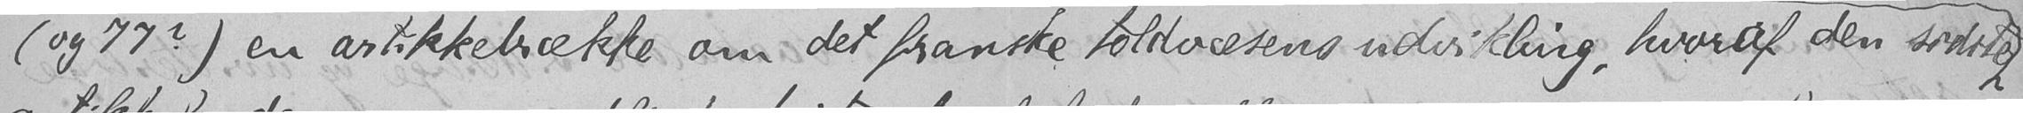(og 77?) en artikkelrække om det franske toldvæsens udvikling, hvoraf den sidste
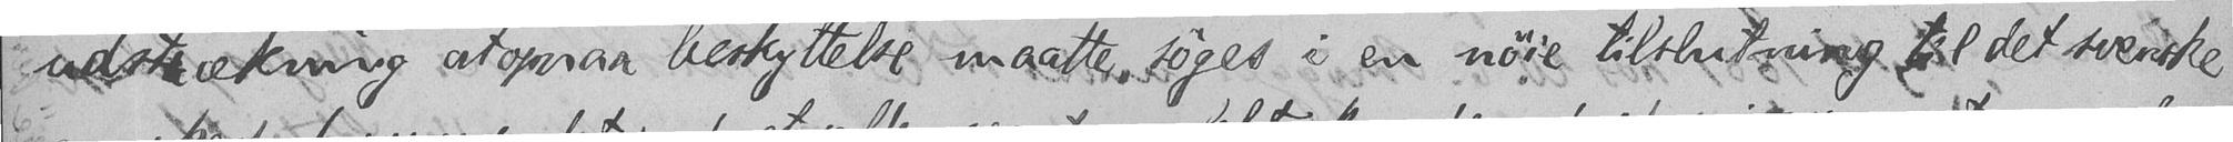udstrækning at opnaa beskyttelse maatte søges i en nøie tilslutning til det svenske
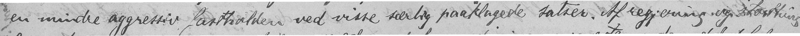en mindre aggressiv fastholden ved visse særlig paaklagede satser. Af regjering og storthing
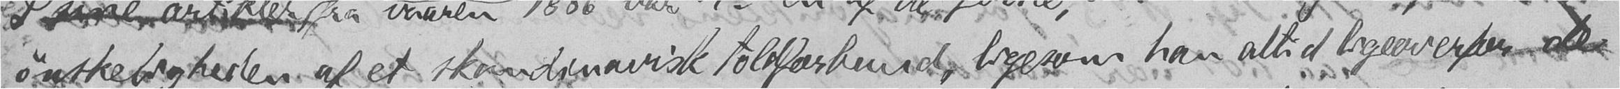ønskeligheden af et skandinavisk toldforbund, ligesom han altid ligeoverfor de
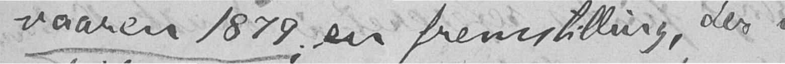vaaren 1879; en fremstilling, der i
